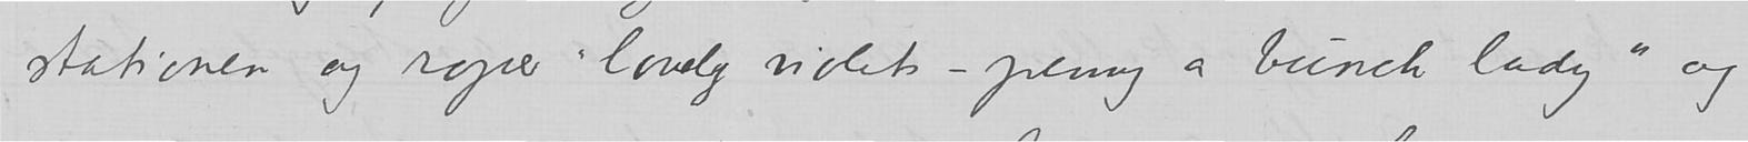stationen og roper «lovely violets-penny a bunch lady» og
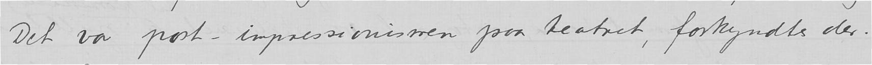– Det var post-impressionismen paa teatret, forkyndtes der.
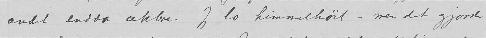andet endda <æklere>. Jeg lo himmelhøit – men det gjorde
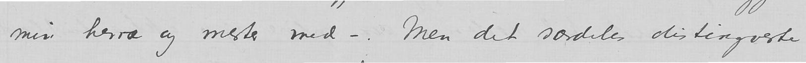min herre og mester med –. Men det særdeles distingverte
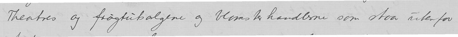Theatres og frugtutsalgene og blomsterhandlerne som staar utenfor
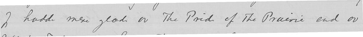Jeg hadde mere glæde av The Pride of the Prairie end av
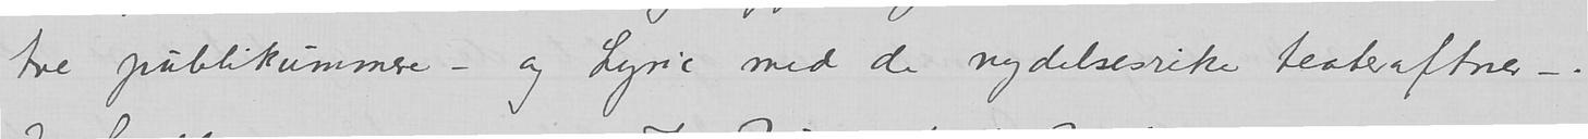eller tre publikummere – og Lyric med de nydelsesrike teateraftner –.
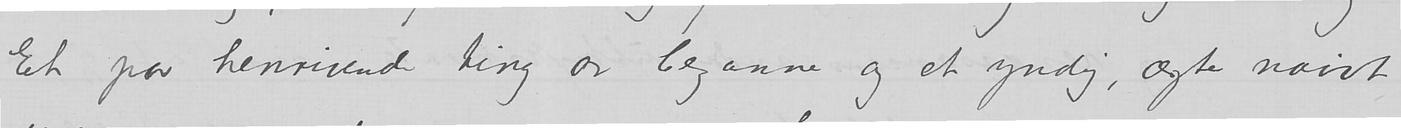Et par henrivende ting av Cezanne og et yndig, ægte naivt
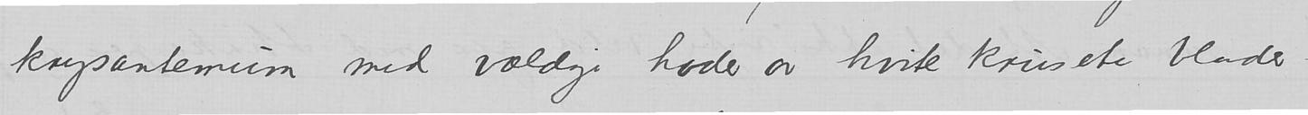krysantenum med vældige hoder av hvite krusete blader –
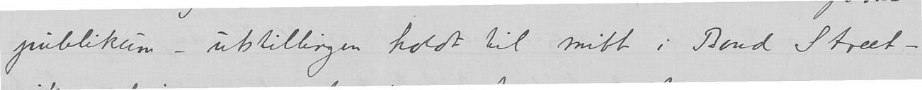publikum – utstillingen holdt til mitt i Bond Street –
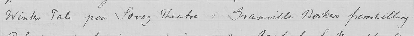Winters Tale paa Savoy Theatre i Granville Barkers fremstilling.
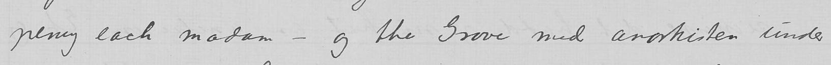penny each madam – og the Grove med anarkisten under
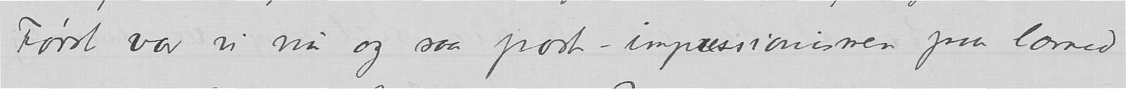Først var vi nu og saa post-impressionismen paa lærred
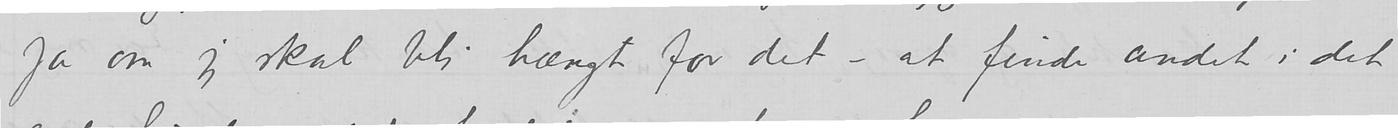Ja om jeg skal bli hængt for det – at finde andet i det
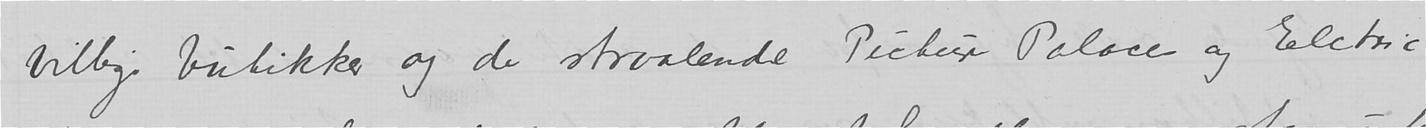billige butikker og de straalende Picture Palace og Electric
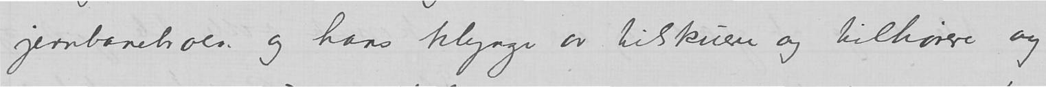jernbanebroen og hans klynge av tilskuere og
